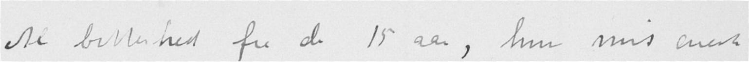Al bitterhed fra de 15 aar, hvor mit eneste
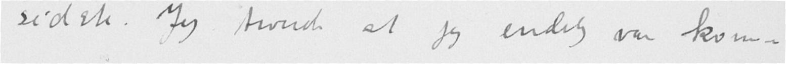sidste. Jeg troede at jeg endelig var kom-
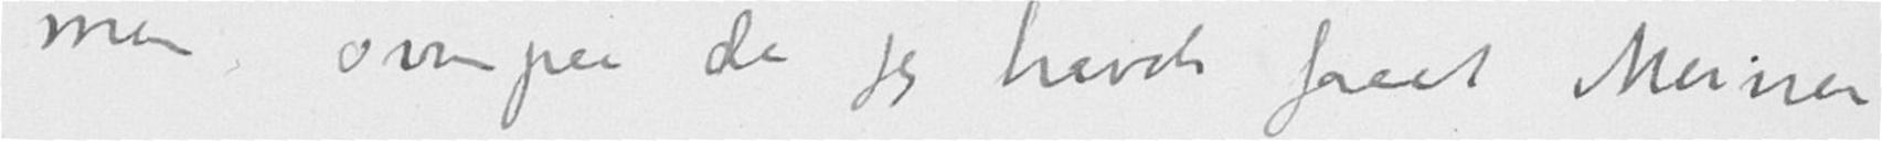men ovenpaa da jeg havde faaet Meiner
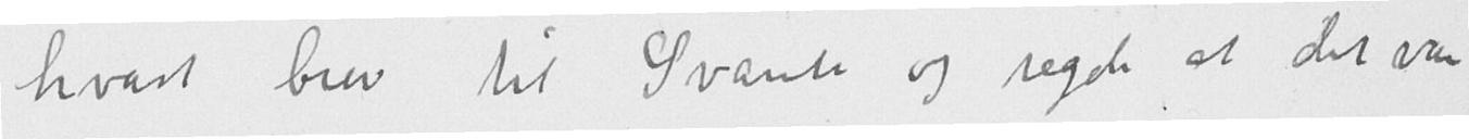krast brev til Svante og sagde at det var
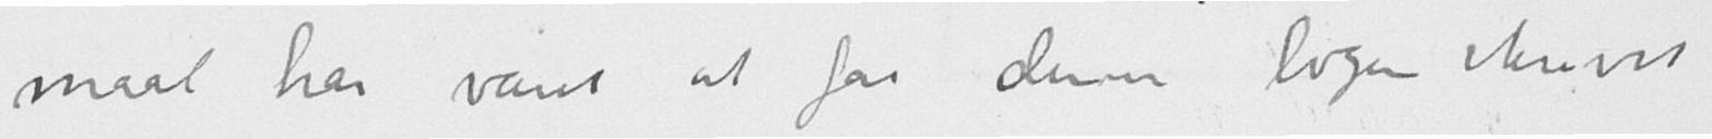maal har været at faa denne bogen skrevet
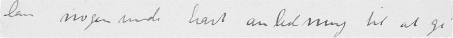lan nogen sinde havt anledning til at gi
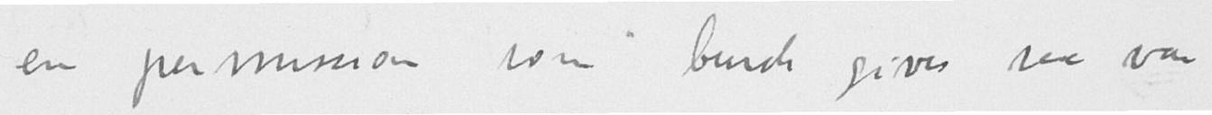en permission som burde gives saa var
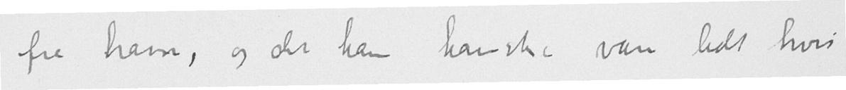fra ham, og det kan kanske være lidt hvis
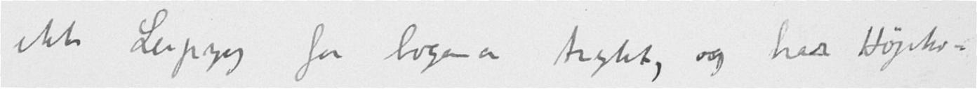ikke Leipzig før bogen er trykt, og har Høgsko-
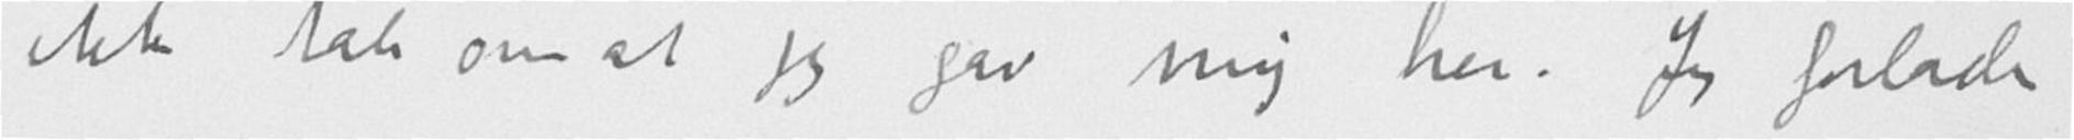ikke tale om at jeg gav mig her. Jeg forlader
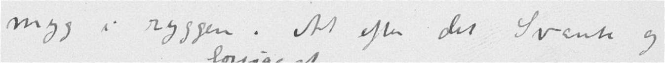mig i ryggen. At efter det Svante og
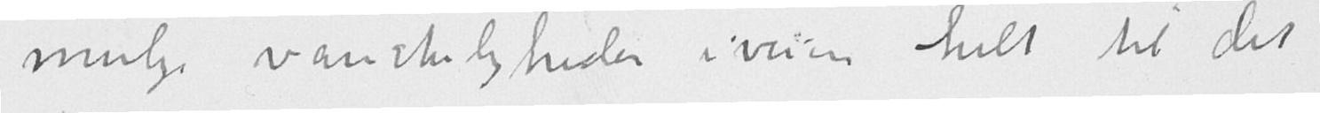mulige vanskeligheder i veien helt til det
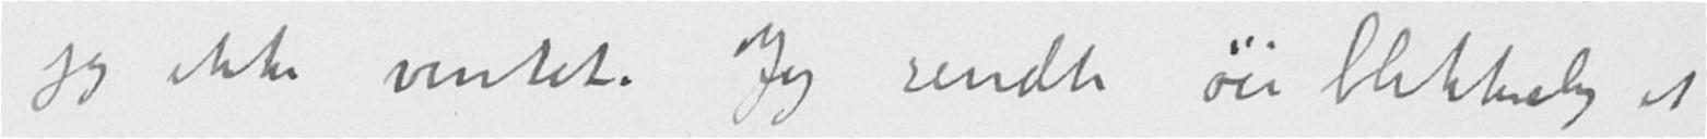jeg ikke ventet. Jeg sendte øieblikkelig et
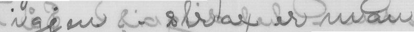igjen, - strax er man
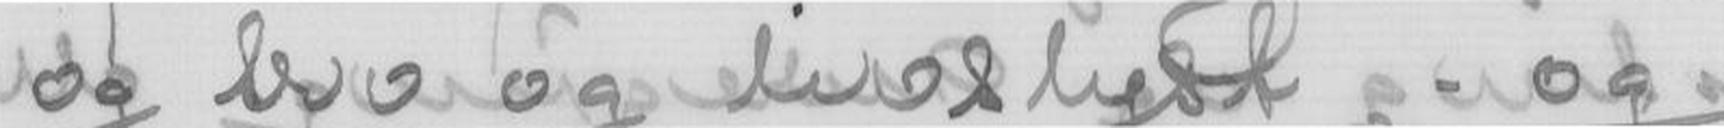og tro og livslyst, - og
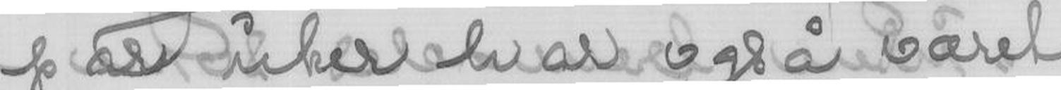par uker har også været
